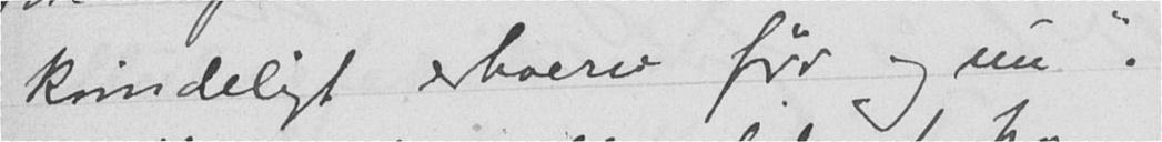kvindeligt erhverv før og nu".
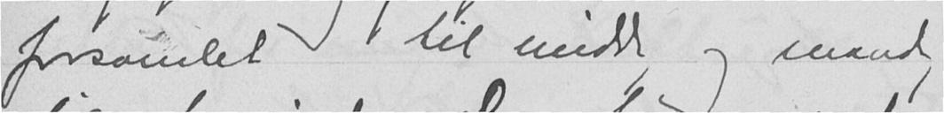forsamlet til middag og mand,
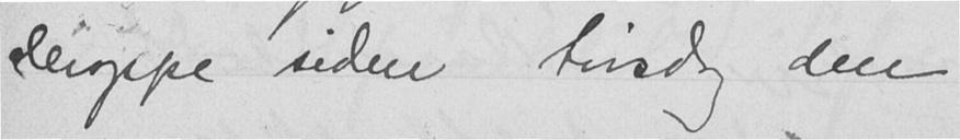deroppe siden tirsdag den
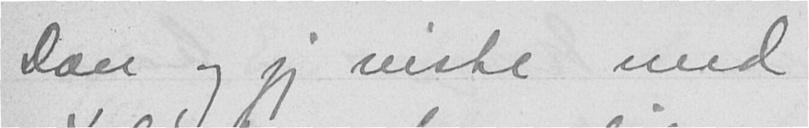Don og jeg reiste med
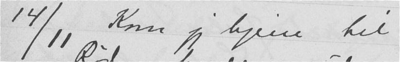14/11 Kom jeg hjem til
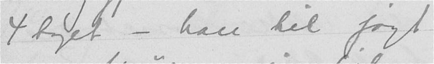4 toget - han til jagt
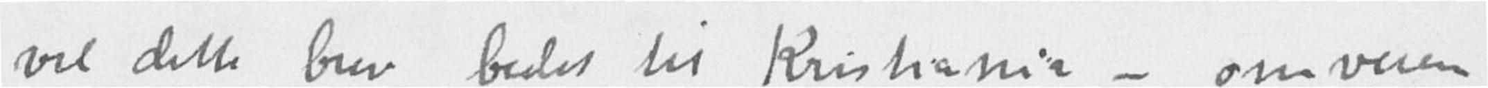vel dette brev bedst til Kristiania - omveien
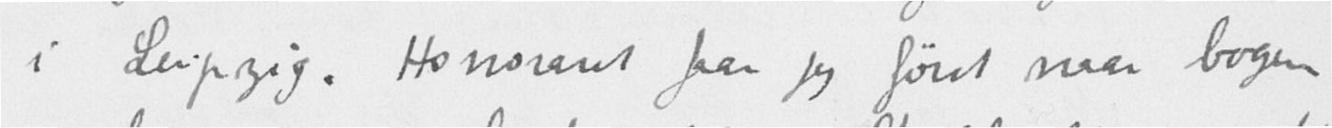i Leipzig. Honoraret faar jeg først naar bogen
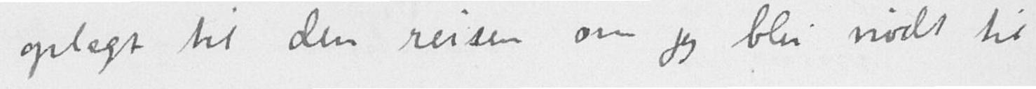oplagt til den reisen om jeg blir nødt til
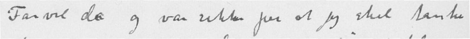Farvel da og vær sikker paa at jeg skal tænke
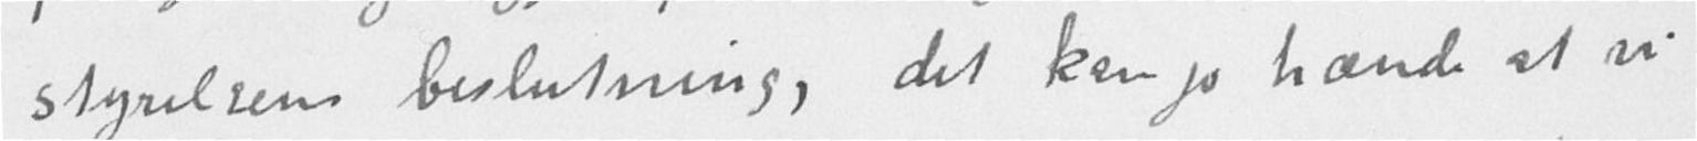styrelsens beslutning, det kan jo hænde at vi
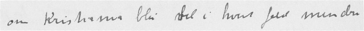om Kristiania blir vel i hvert fald mindre
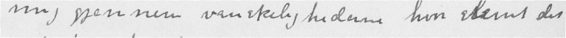mig gjennem vanskelighederne hvor slemt det
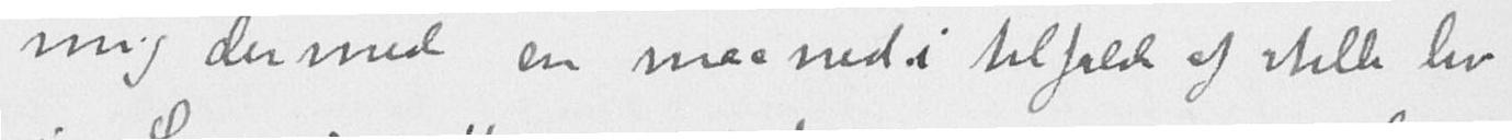mig dermed en maaned i tilfald og stille liv
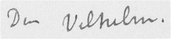Din Vilhelm.
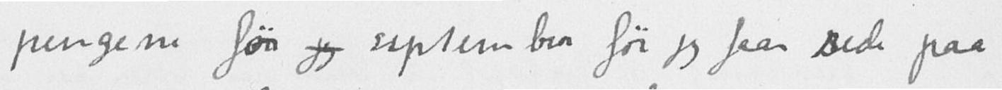pengene før jeg september før jeg faar rede paa
